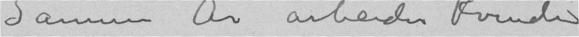Samme År arbeider Kvinden
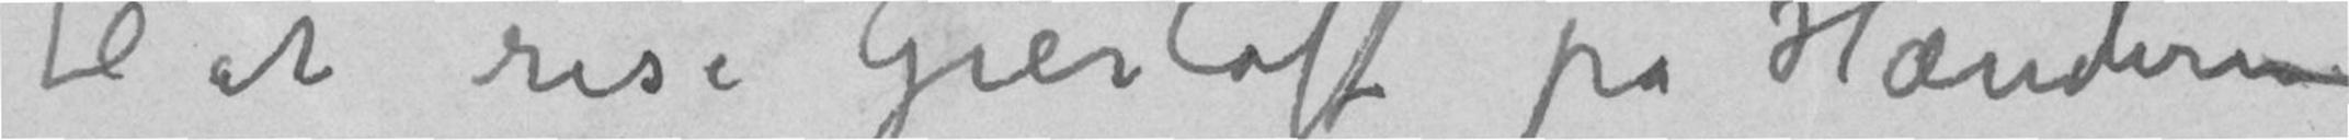til at rise Gierløff på Hænderne
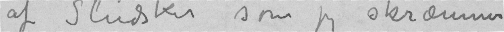af Sludsker som jeg skræmmer
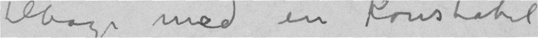tilbage med en Konstabel
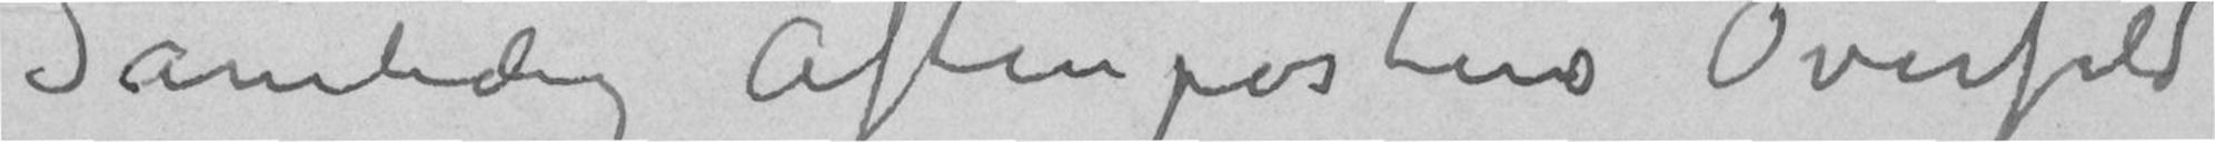Samtidig Aftenpostens Overfald
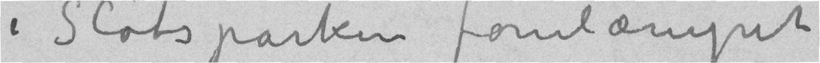i Slotsparken forulæmpet
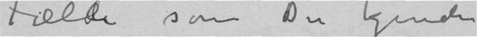Fælde som du kjender
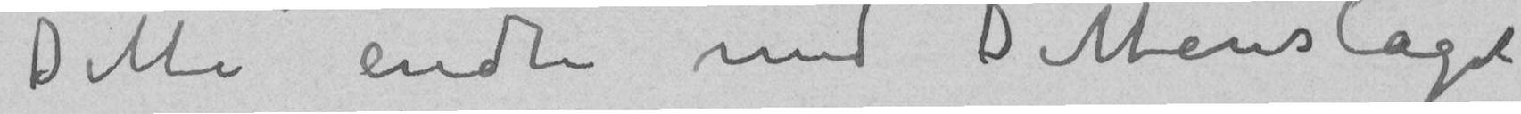Dette endte med Dittenslaget
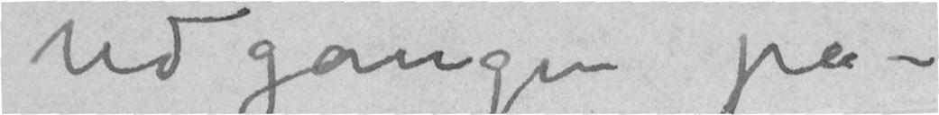udgangen på –
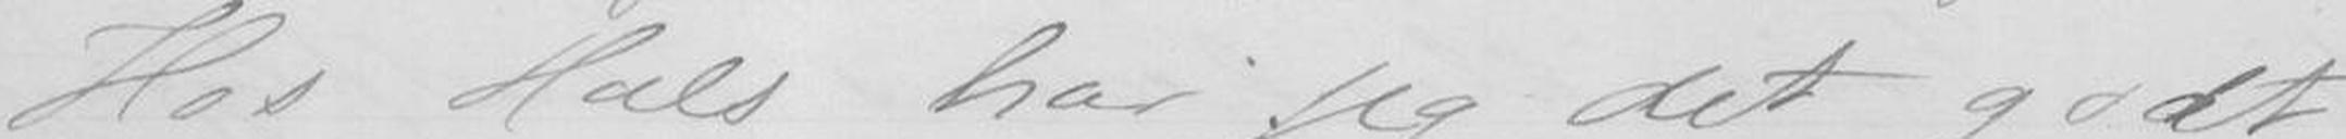Hos Hals har jeg det godt,
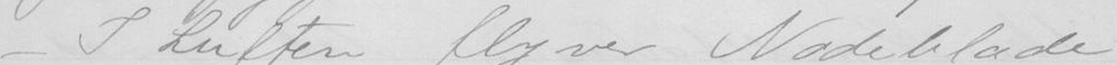- I Luften flyver Nodeblade,
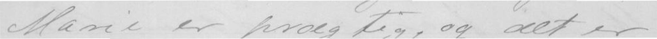Marie er prægtig, og det er
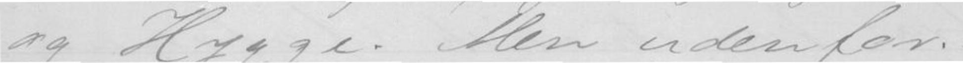og Hygge. Men udenfor. -
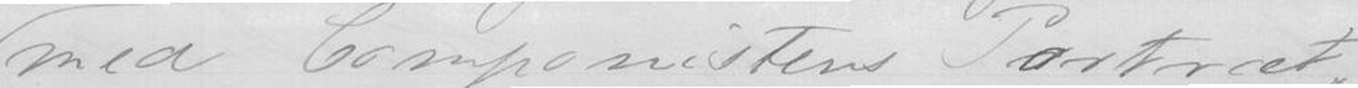de sidste med Componistens Portræt,
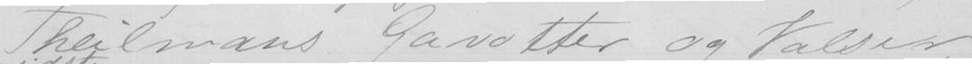Theilmans Gavotter og Valser,
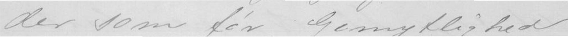der som før, Gemytlighed
